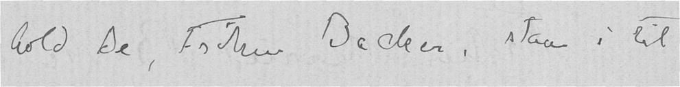hold De, Frøken Backer, staar i til
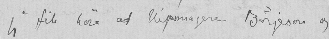jeg fik høre at Gipsmagere Bøjeson og
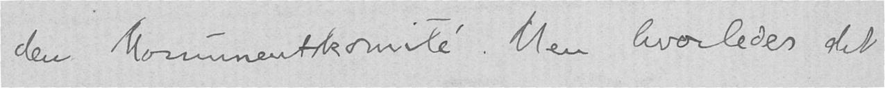den Monumentkomite. Men hvorledes det
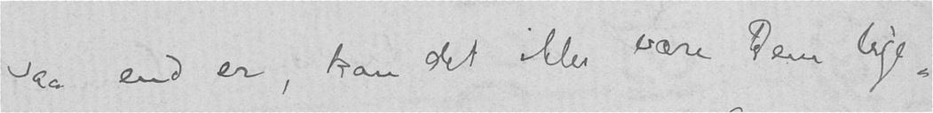saa end er, kan det ikke være Dem lige-
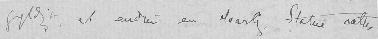gyldigt, at endnu en daarlig Statue sættes
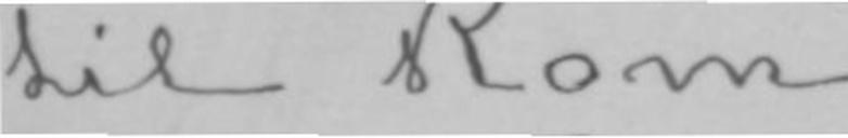til Rom.
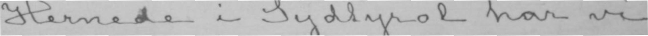Hernede i Sydtyrol har vi
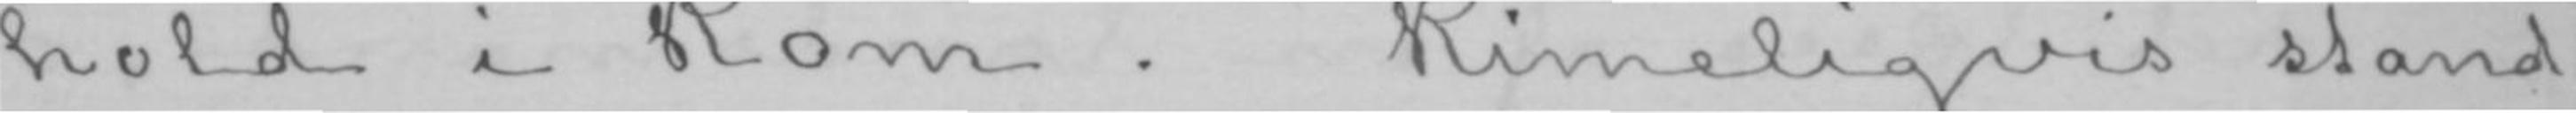hold i Rom. Rimeligvis stand-
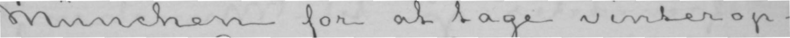München for at tage vinterop-
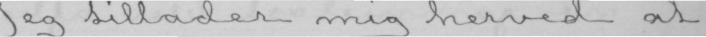Jeg tillader mig herved at
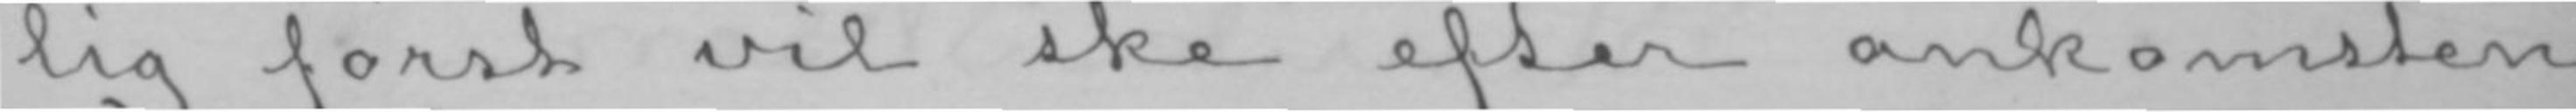lig først vil ske efter ankomsten
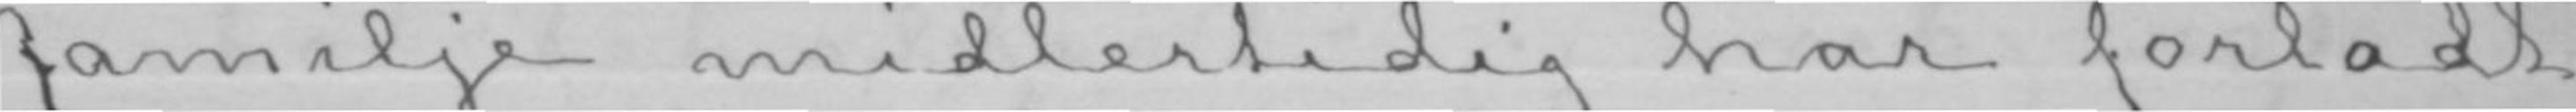familje midlertidig har forladt
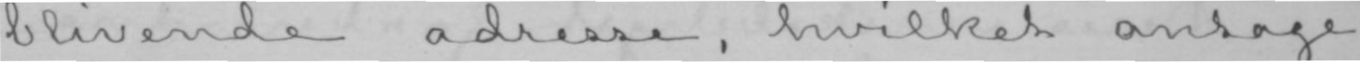blivende adresse, hvilket antage-
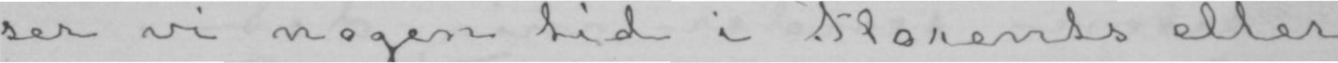ser vi nogen tid i Florents eller
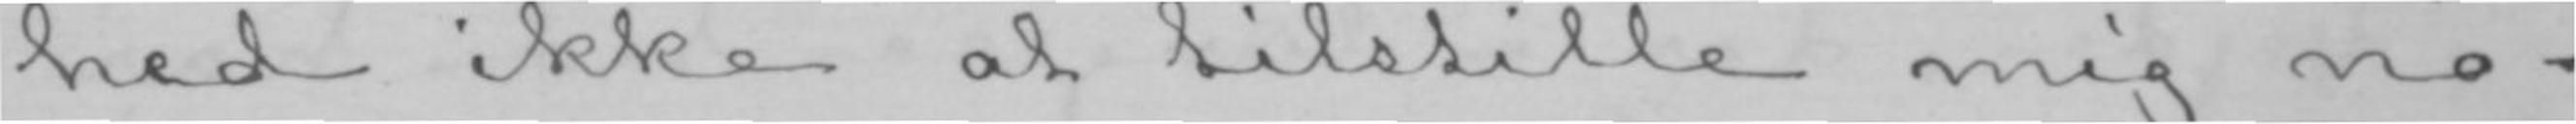hed ikke at tilstille mig no-
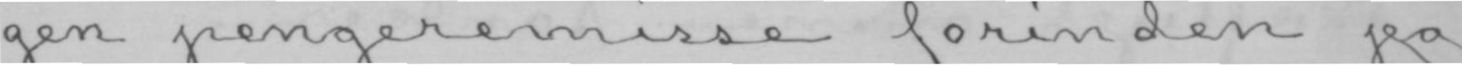gen pengeremisse forinden jeg
